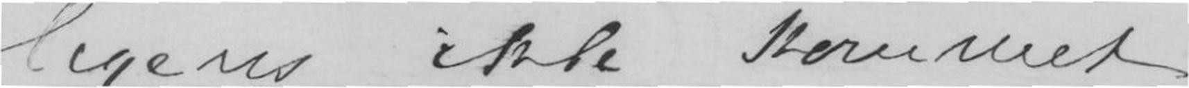ligens ikke kommet
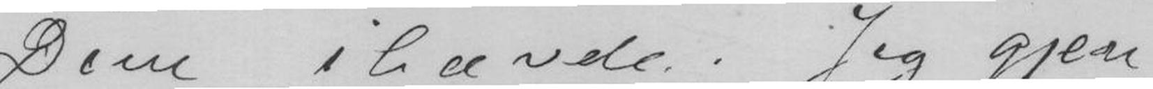Dem ihænde. Jeg gjen-
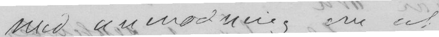med anmodning om at
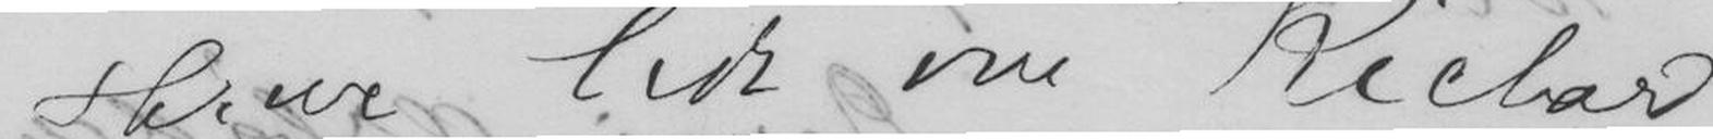skrive lide om Richard
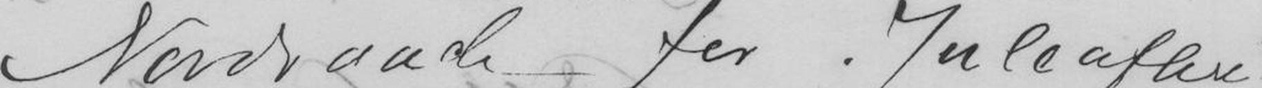Nordraach for "Julaften"
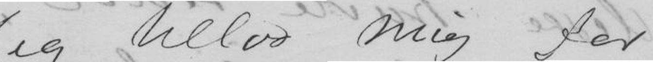Jeg tillod mig for
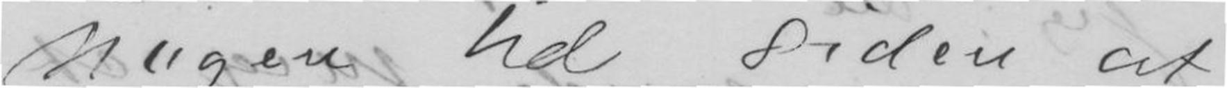Nogen tid siden at
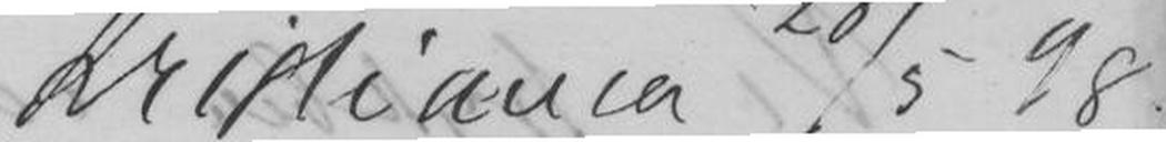Kristiania 28/5 98
